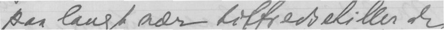paa langt nær tilfredstiller de
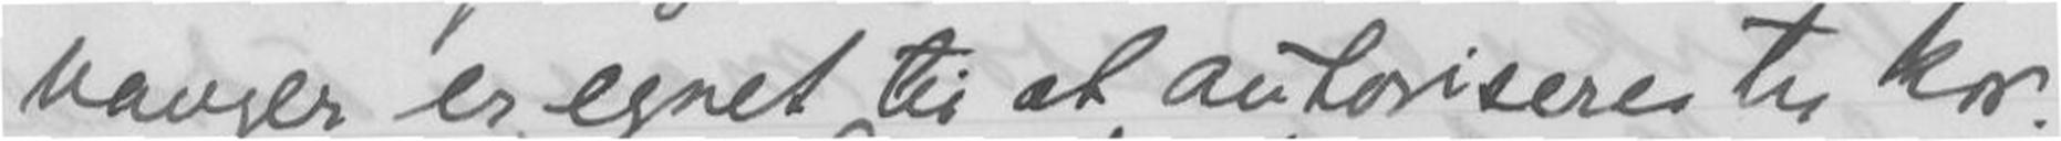vanger er egnet til at autoseres til Kir-
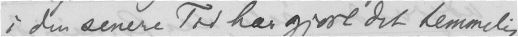i den senere Tid har gjort det temmelig
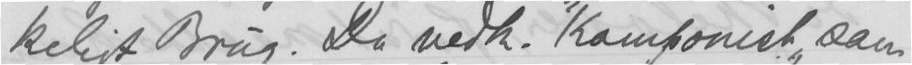keligt Brug. Da vedk. "Komponist" som
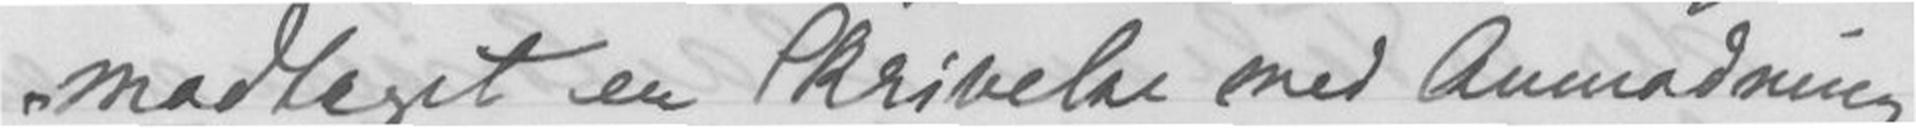modtaget en Skrivelse med Anmodning
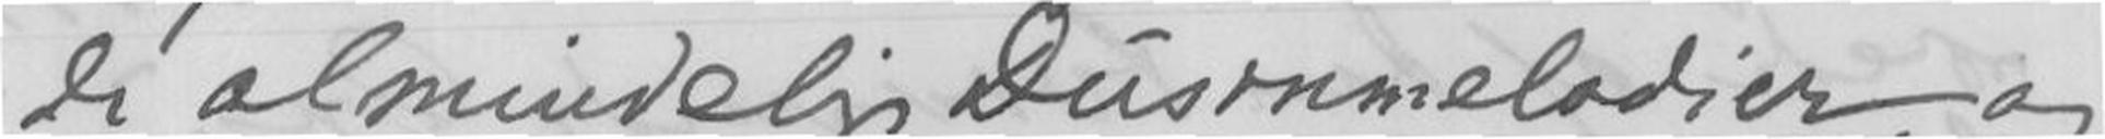de almindelige Dusinmelodier,
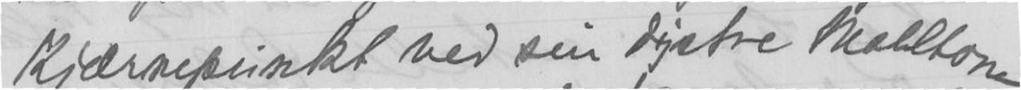kjærnepunkt ved sin dystre Molltone
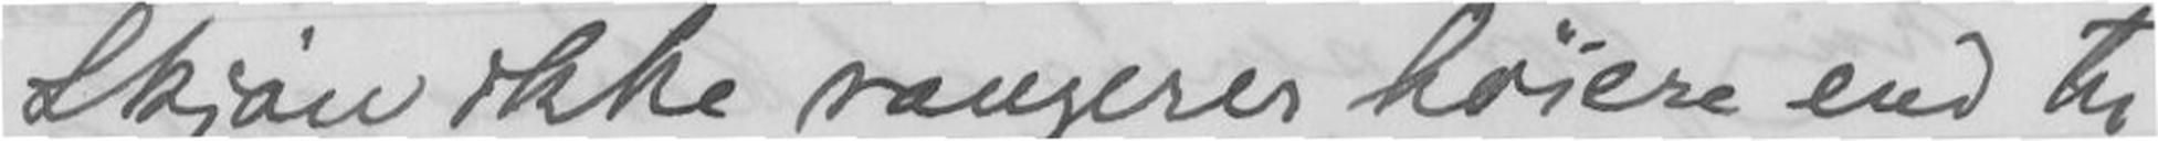Skjøn ikke rangerer høiere end til
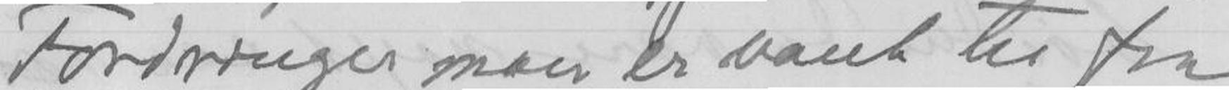Fordringer man er vant til fra
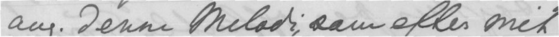ang. denne Melodi, som efter mit
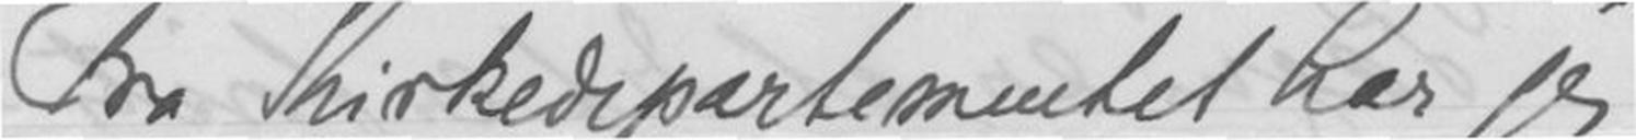Fra Kirkedepartementet har jeg
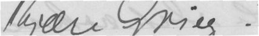Kjære Grieg!
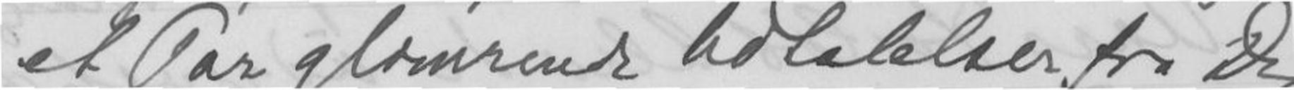et Par glimrende Udtalelser fra Dig
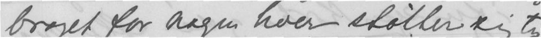broget for nogen hver støtter sig til
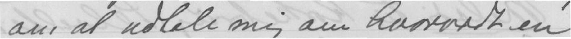om at udtale mig om hvorvidt en
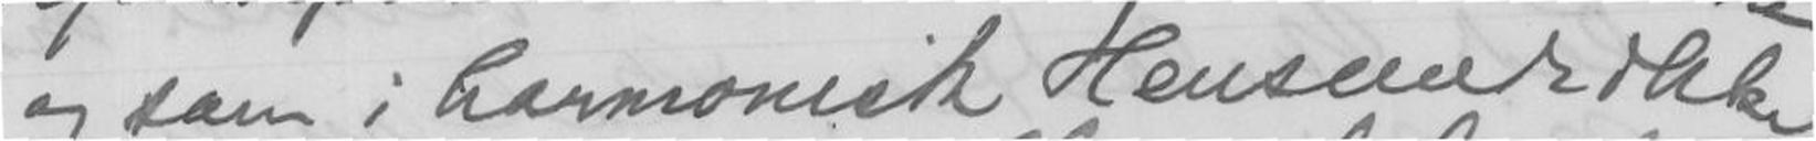og som i harmonisk Henseende ikke
